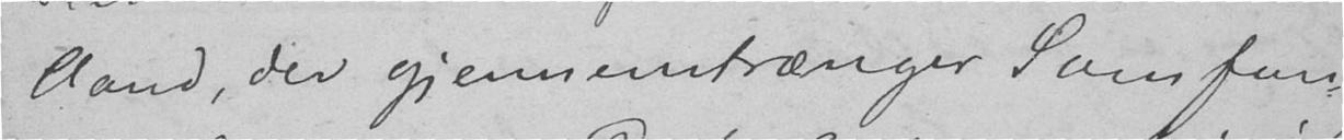Aand, der gjennemtrænger Samfun-
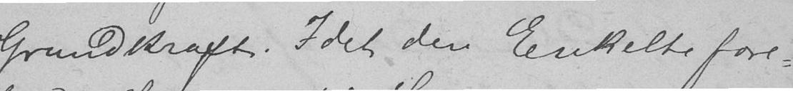Grundkraft. Idet den Enkelte fore-
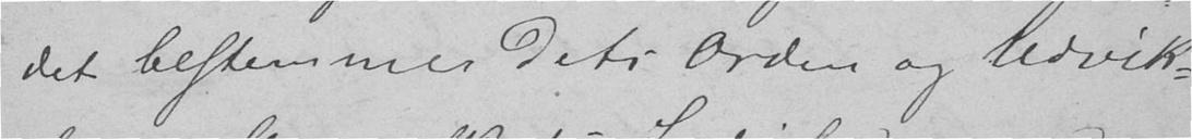det bestemme des Orden og Udvik-
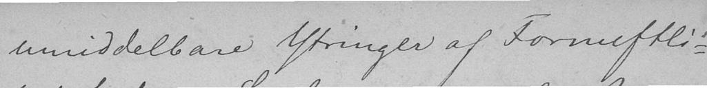umiddelbare Ytringer af Fornuftli-
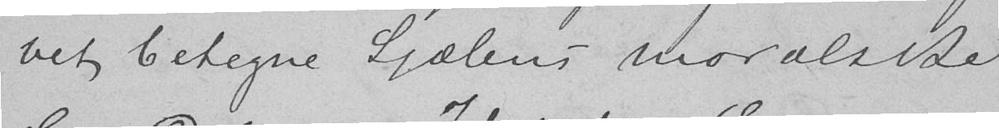vet betegne Sjælens moralske
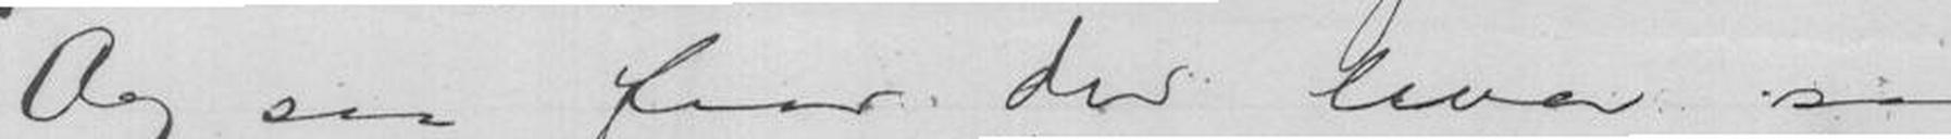Og saa faar Du leve saa
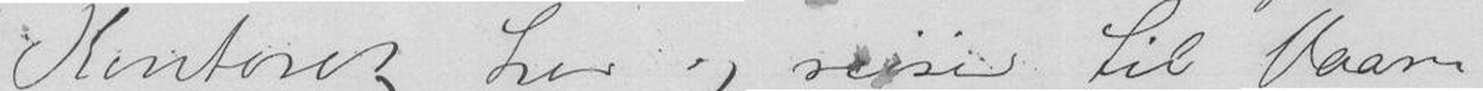Kontoret her og reiser til Vaaren
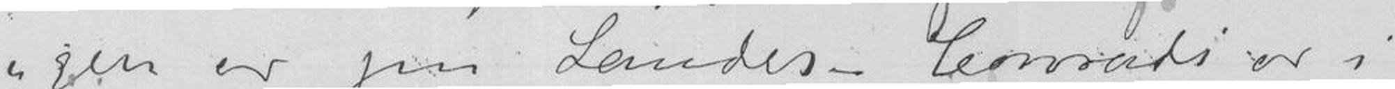gen er paa Landet - Conradi er i
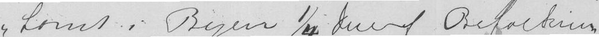tomt i Byen 1/4 deraf Befolknin-
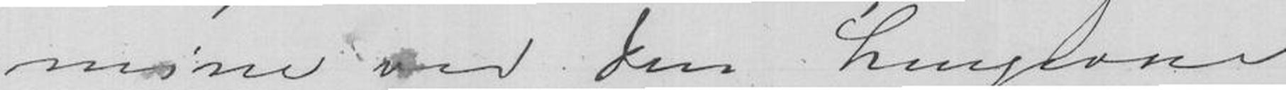mine ved Din hengivne
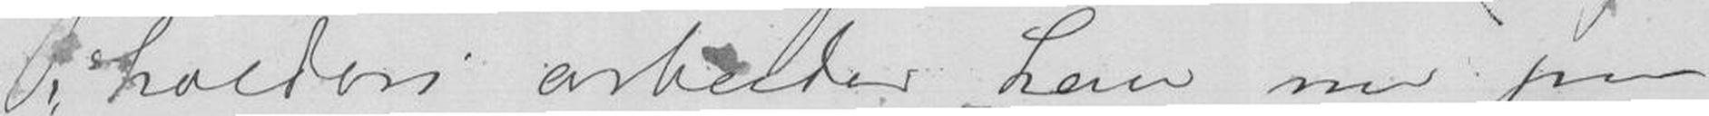holderi arbeider han nu paa
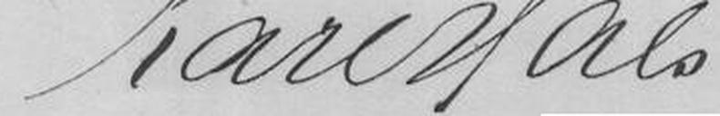Karl Hals
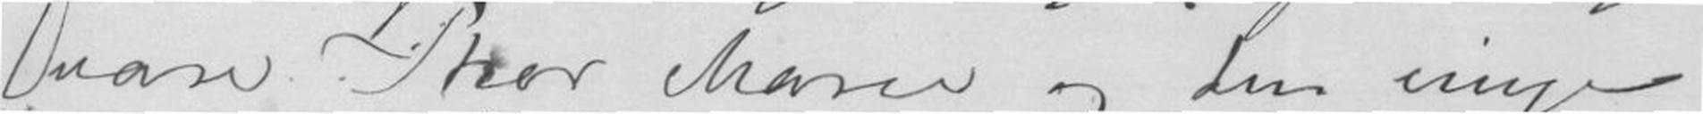vare Thor Marie og den unge
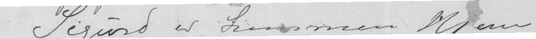Sigurd er kommen Hjem
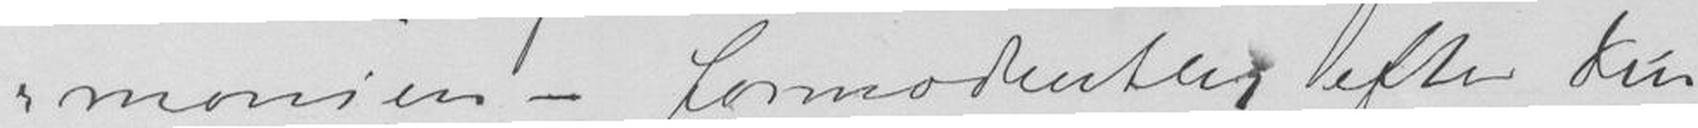monien - formodentlig efter Din
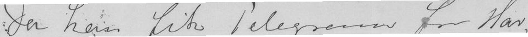Da han fik Telegram fra Har-
